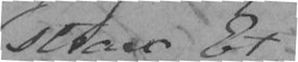strax Et. -
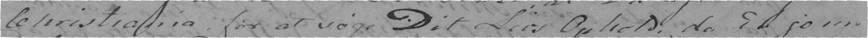Christiania for at søge Dit Livs Ophold, da En jo nu
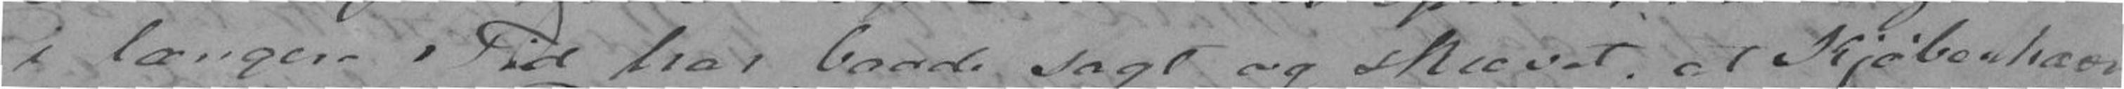i længere Tid har baade sagt og skrevet at Kjøbenhavn
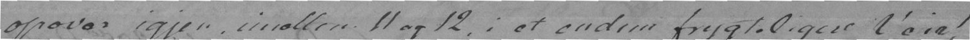opover igjen imellem 11 og 12, i et endnu frygteligere Veir!
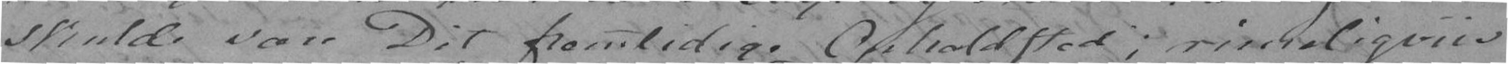skulde være Dit fremtidige Opholdsted; rimeligviis
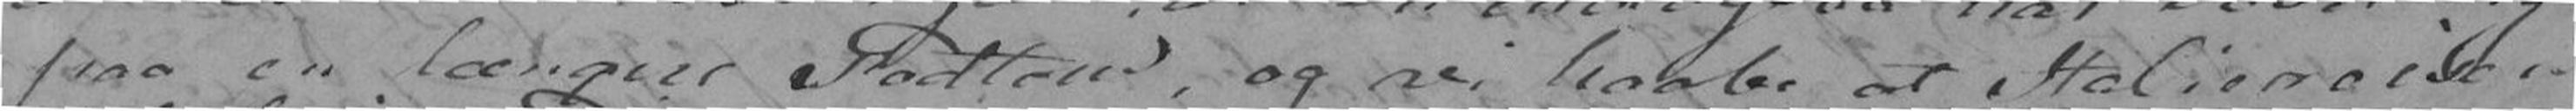paa en længre Fodtour, og vi haaber at Italiereisen
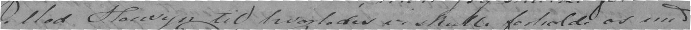Mod Hensyn til hvorledes vi skulde forholde os med
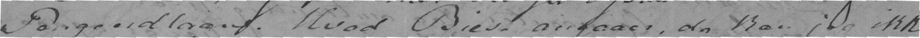Pengelaan. Hvad Biese angaar, da kan jeg ikke
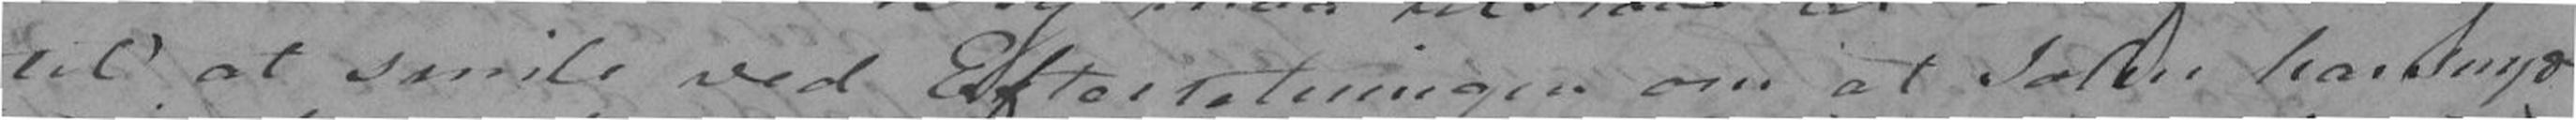til at smile ved Efterretningen om at John har snydt
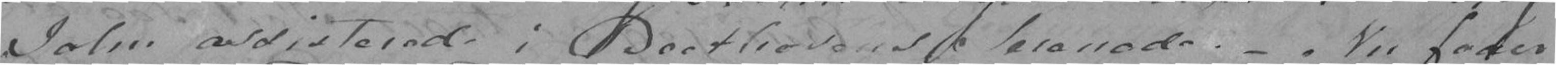John assisterede i Beethovens Serenade. - Nu faaer
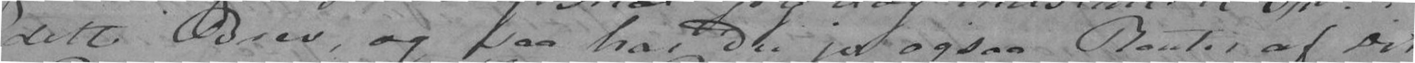dette Brev, og saa har Du jo ogsaa Renter af Dit
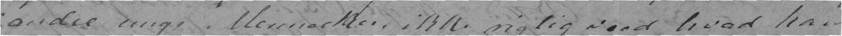andre unge Mennesker ikke rigtig veed hvad han
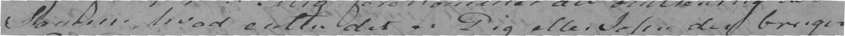Samme, hvad endten det er Dig eller John der bruger
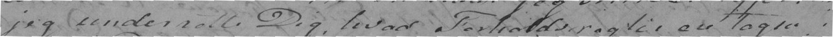jeg underrette Dig, hvad Forholdsregler ere tagen i
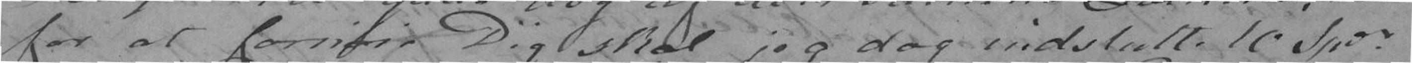for at fornøie Dig skal jeg dog indslutte 10 Spdo i
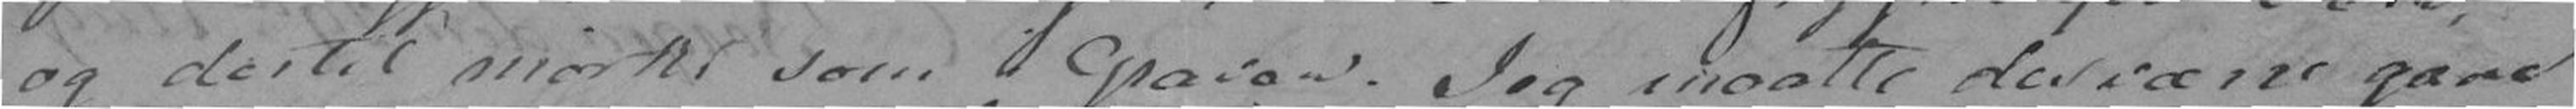og dertil mørkt som i Graven. Jeg maatte desværre gaae
